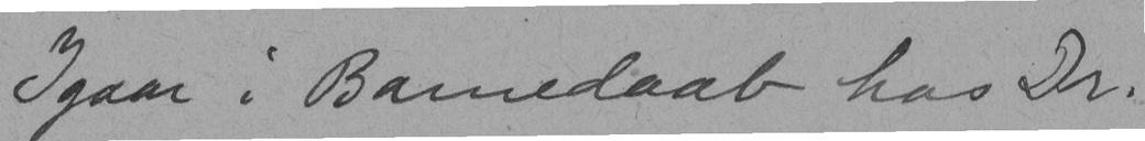Igaar i Barnedaab hos Dr.
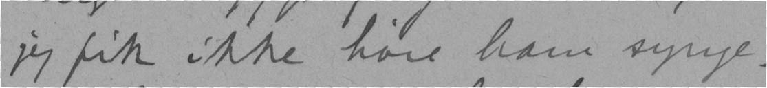jeg fik ikke høre ham synge.
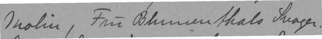Molin, Fru Blumenthals Svoger.
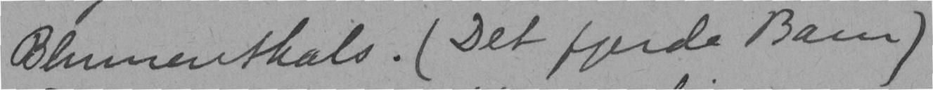Blumenthals. (Det fjerde Barn)
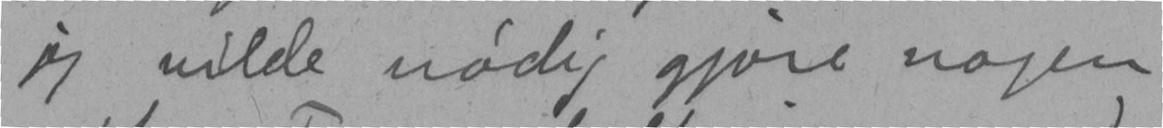jeg vilde nødig gjøre nogen
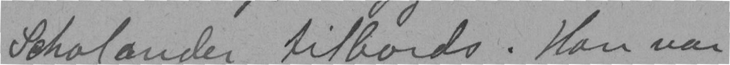Scholander tilbords. Han var
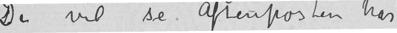De vil se Aftenposten har
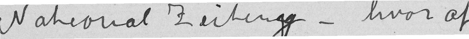National Zeitung – hvoraf
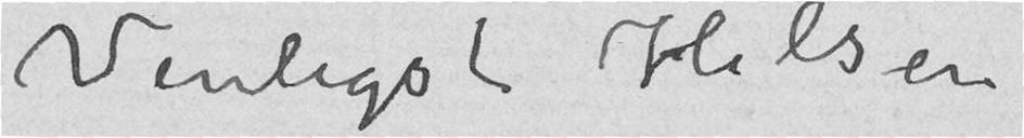Venligst Hilsen
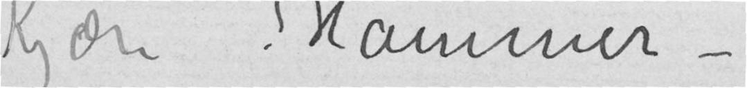Kjære Hammer –
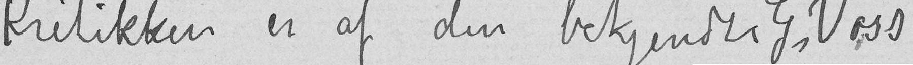Kritikken er af den bekjendte G. Voss
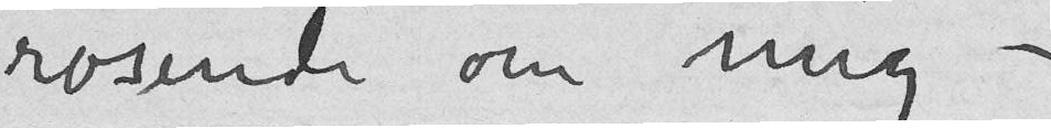rosende om mig –
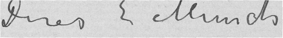Deres E Munch
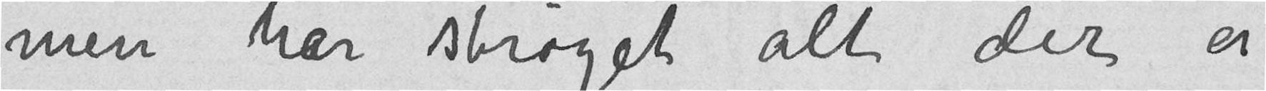men har strøget alt der er
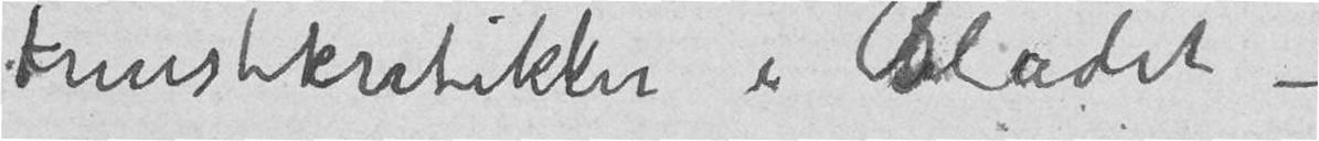kunstkritiker i Bladet –
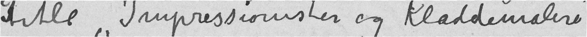Title «Impressionister og Kladdemalere»
